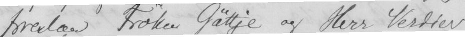Frøken Gättje og Herr Verdler
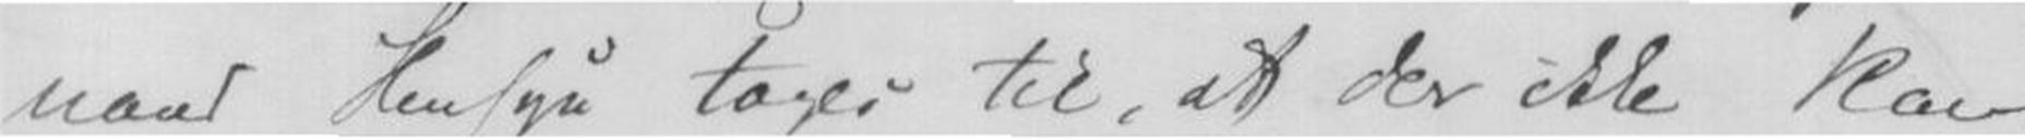naar tager til, at der ikke kan
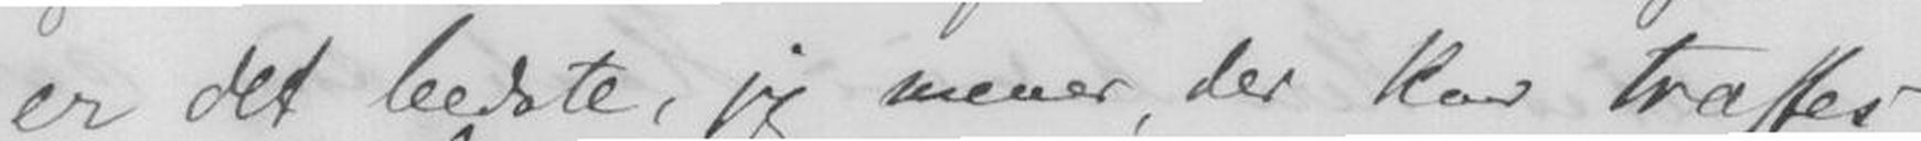er det bedste, jeg mener, der kan træffes
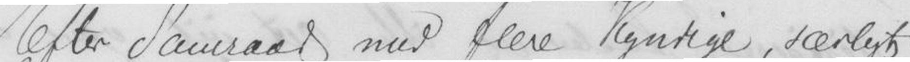Efter Samraad med flere Kyndige, særligt
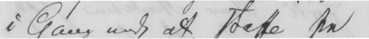i Gang med at skaffe fra
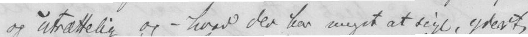og utrættelig og - hvad der har meget at sige,
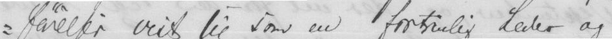førellser vist sig som en fortrolig og
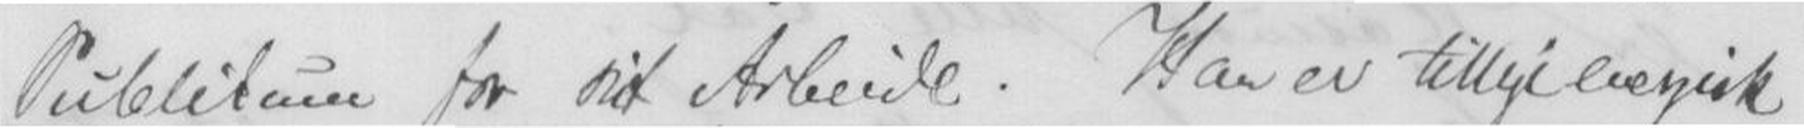Publikum for sit arbeide. Han er tillige
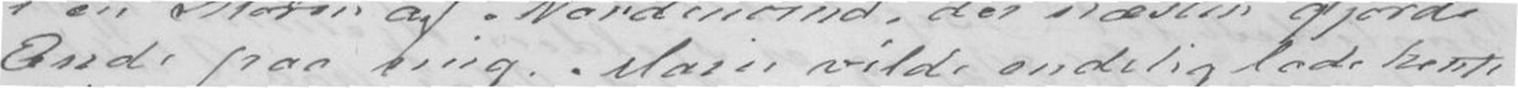Ende paa mig: Marie vilde endelig lade hente
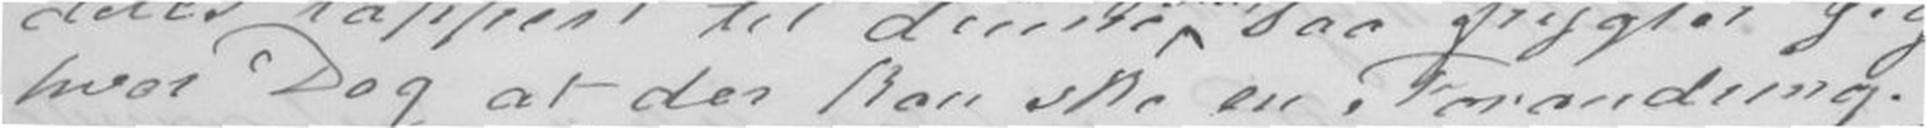hver Dag at der kan ske en Forandring.
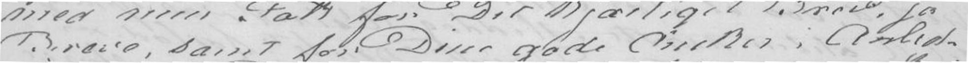Breve, samt for Dine gode Ønsker saa skrø-
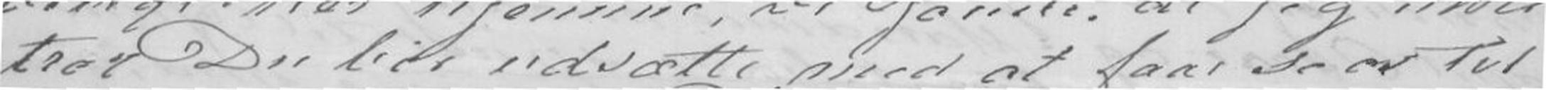tror Du bør udsætte med at faae se os til
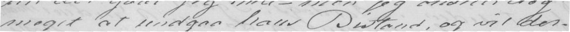meget at undgaa hans Bistand, og vil der-
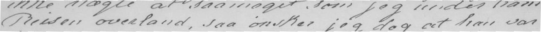Reisen overland, saa ønsker jeg dog at han var
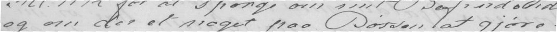og om der et noget paa Børsen at gjøre -
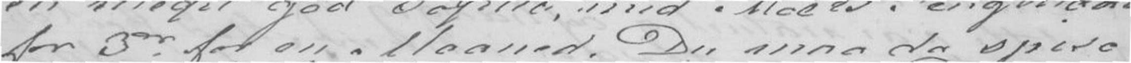for 3do for en Maaned. Du maa da spise
